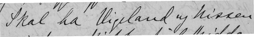Skal ha Vigeland og Nissen
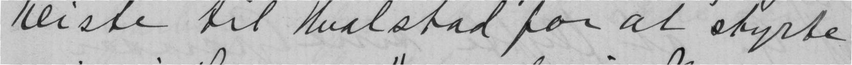reiste til Hvalstad for at styrte
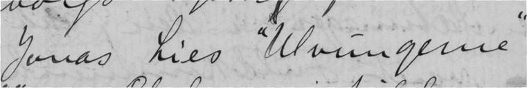Jonas Lies «Ulvungerne"
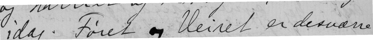idag. Føret og Veiret er desværre
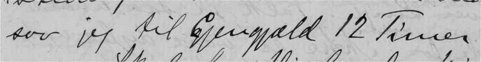sov jeg til Gjengjæld 12 Timer.
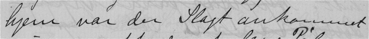hjem var der Slagt ankommet
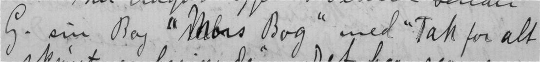G. sin Bog "Mors Bog" med "Tak for alt
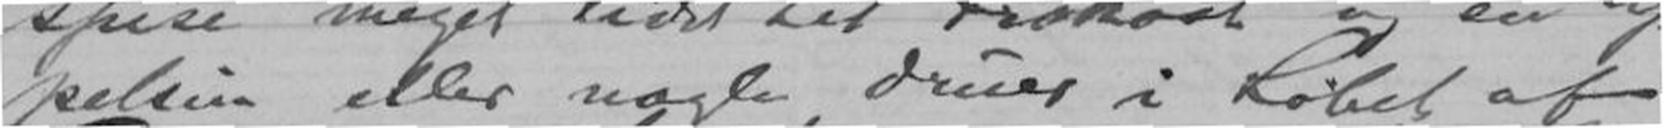pelsin eller nogle Druer i Løbet af
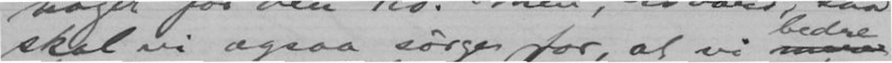skal vi ogsaa sørge for, at vi
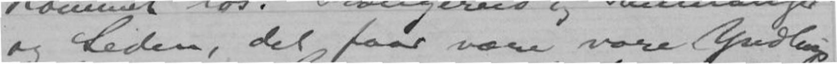og Leden, det faar være vore Yndlings-
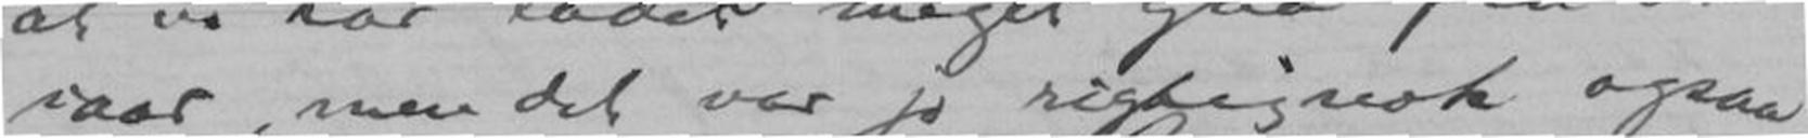iaar, men det var jo rigtignok ogsaa
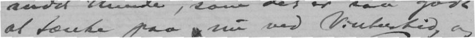at tænke paa nu ved Vintertid, og
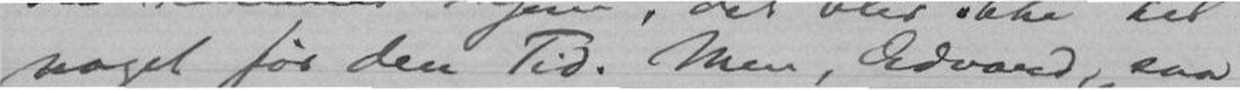noget før den Tid. Men, Edvard, saa
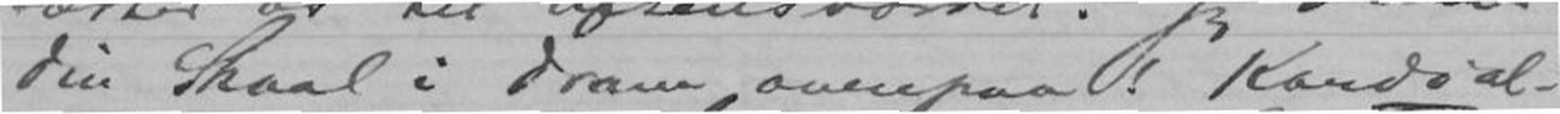Din Skaal i Dram ovenpaa! Kardial-
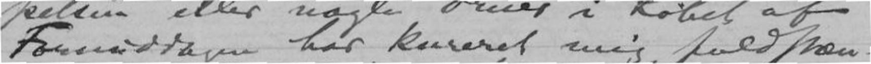Formiddagen har kureret mig fuldstæn-
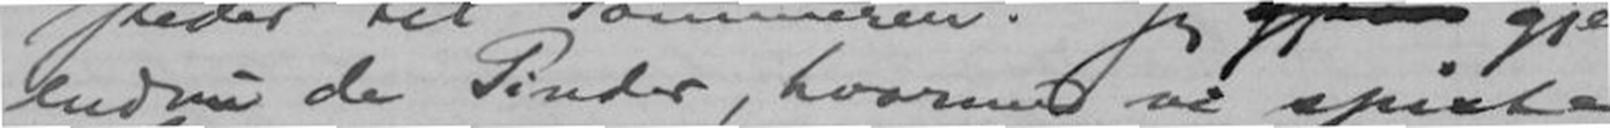endnu de Pinder, hvormed vi spiste
